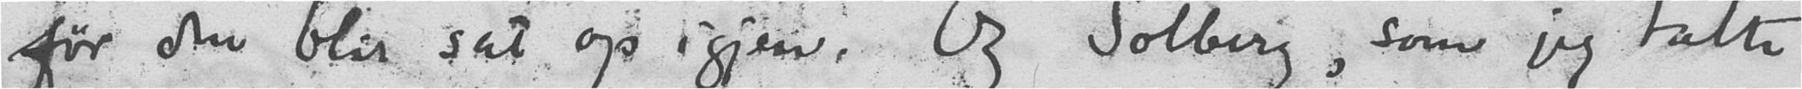før den blir sat op igjen. Og Solberg, som jeg talte
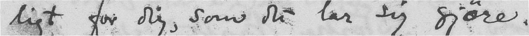ligt for dig, som det lar sig gjøre.
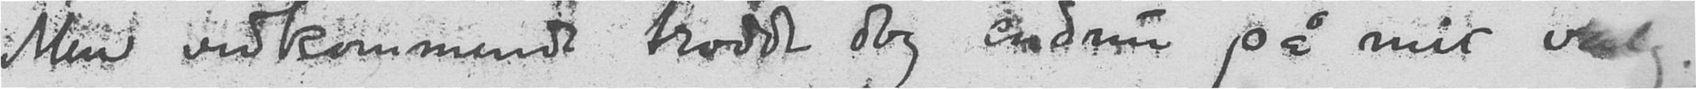Men vedkommende trodde dog endnu på mit valg.
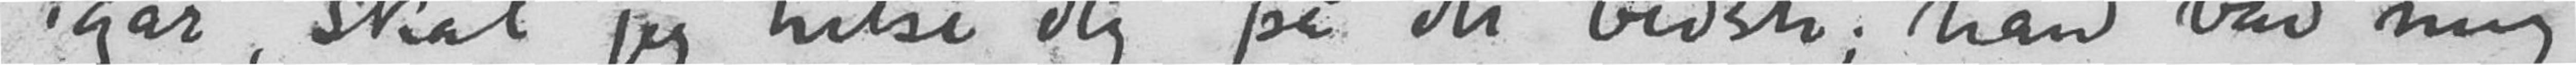igår, skal jeg hilse dig på det bedste; han bad mig
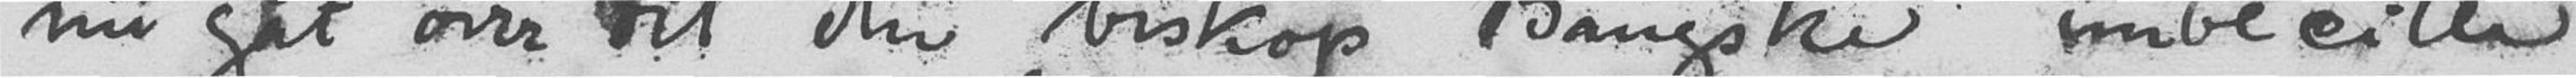nu gåt over til den biskop Bangske imbecille
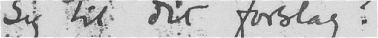sig til dit forslag?
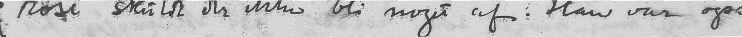rose skulde der ikke bli noget af. Han var også
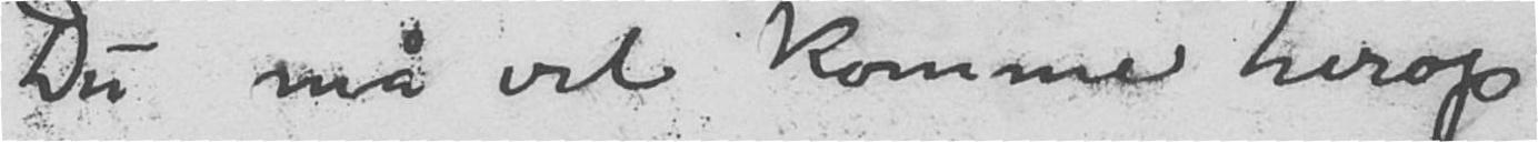Du må vel komme herop
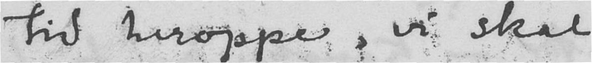tid heroppe, vi skal
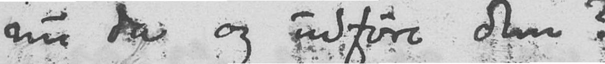nu da og udføre dem?
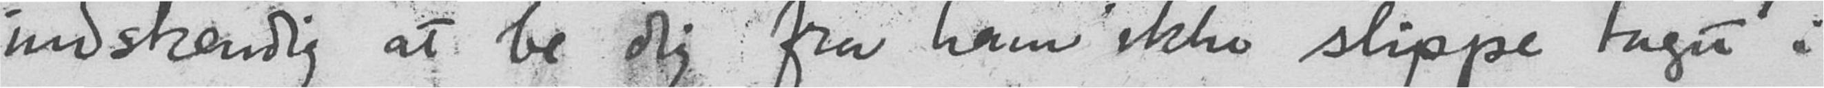indstendig at be dig fra ham ikke slippe taget i
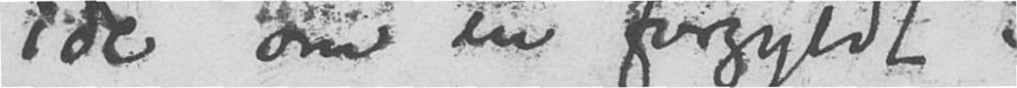ide om en forgyldt
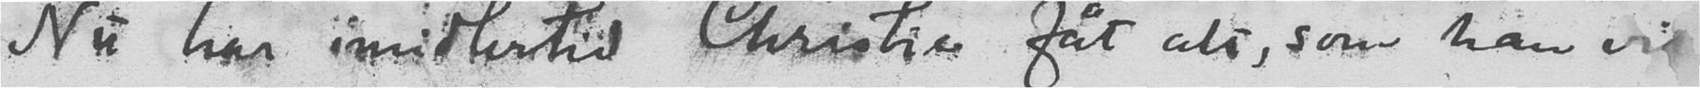Nu har imidlertid Christie fåt alt, som han vil
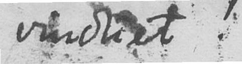vinduet!
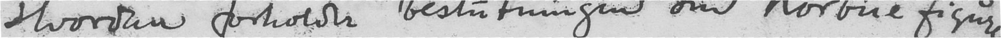Hvordan forholder beslutningen om korbue figurene
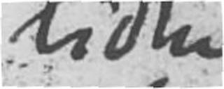liden
